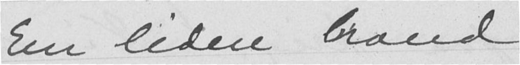En liden brand
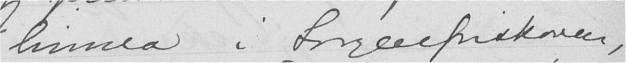linnea i Sorgenfriskoven,
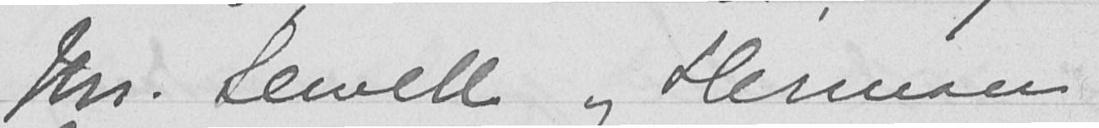Mr. Lewell og Herman
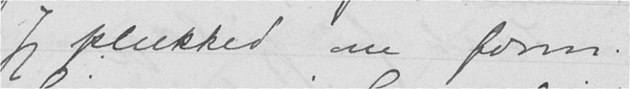Jeg plukked om form.
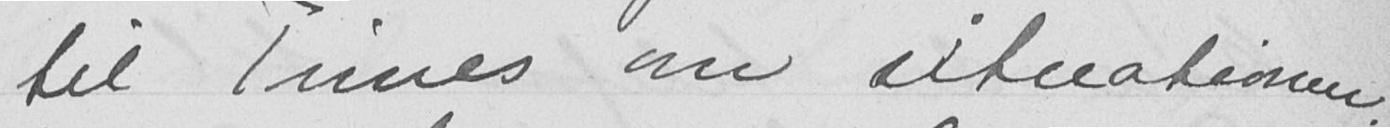til Times om situationen.
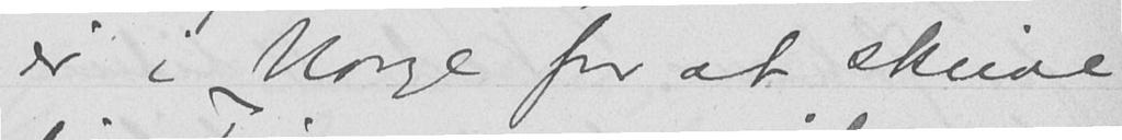er i Norge for at skrive
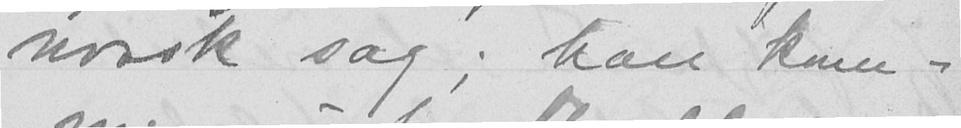norsk sag; han kom-
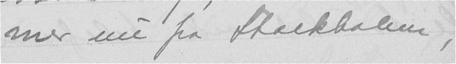mer nu fra Stockholm,
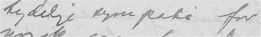tydelige sympati for
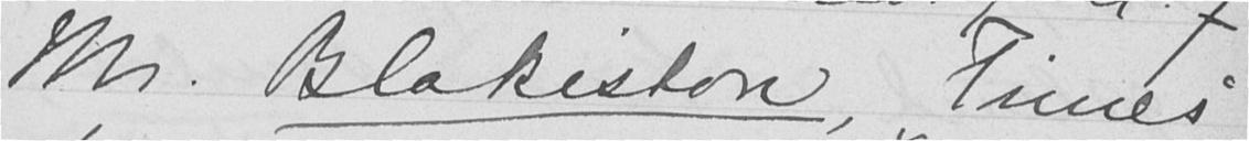Mr. Blakistone, "Times"
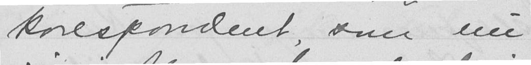korespondent, som nu
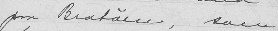paa Bratøen, som
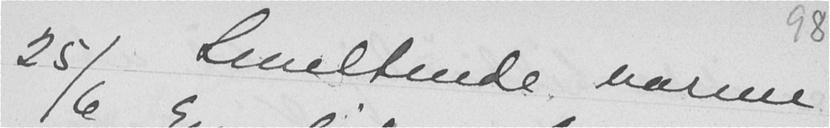25/6 Smeltende varme.
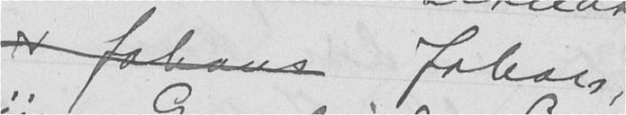er Johans Johan,
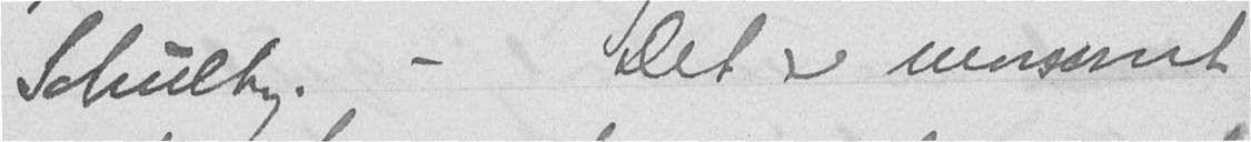Schultz. - Det er morsomt
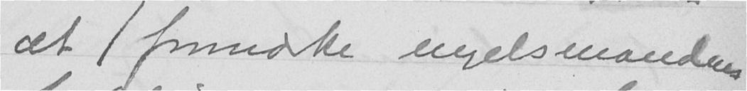at formærke engelsmandens
